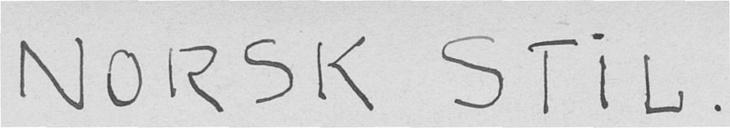NORSK STIL.
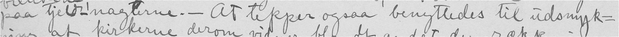paa tjeld-nagterne. - At tepper ogsaa benyttedes til udsmyk-
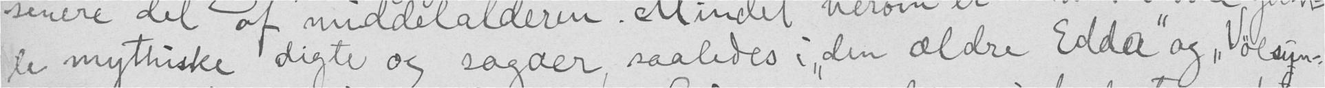le mythiske digte og sagaer, saaledes i "den ældre Edda" og "Vølsun-
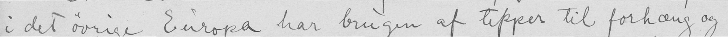i det øvrige Europa har brugen af tepper til forhæng og
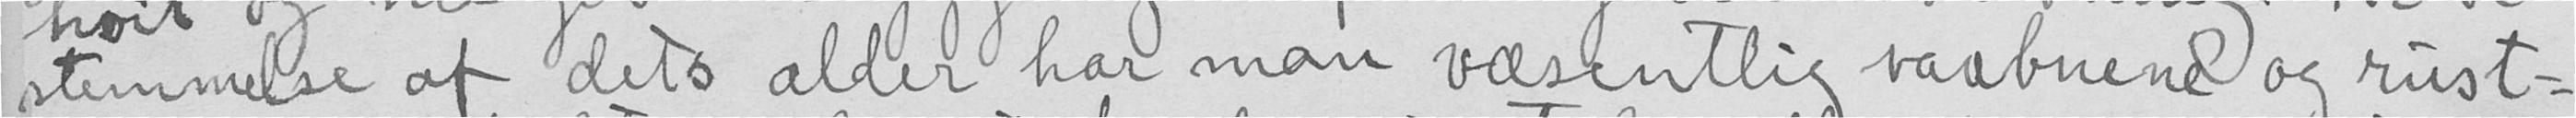stemmelse af dets alder har man væsentlig vaabnene og rust-
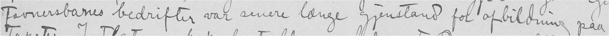Favnersbanes bedrifter var senere længe gjenstand for afbildning paa
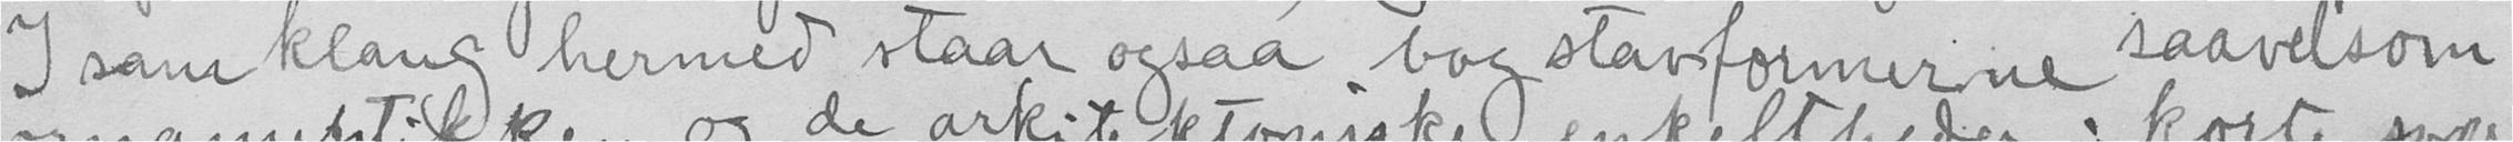I samklang hermed staar ogsaa bogstavformerne saavelsom
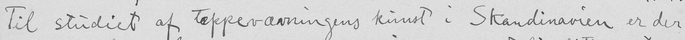Til studiet af teppevævningens kunst i Skandinavien er der
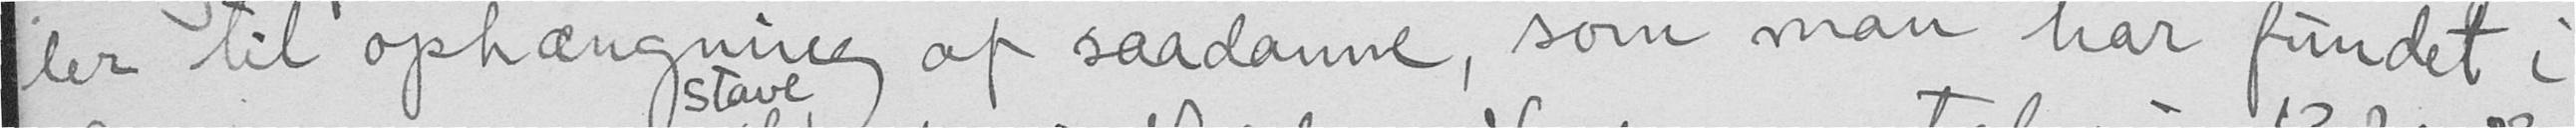ler til ophængning af saadanne, som man har fundet i
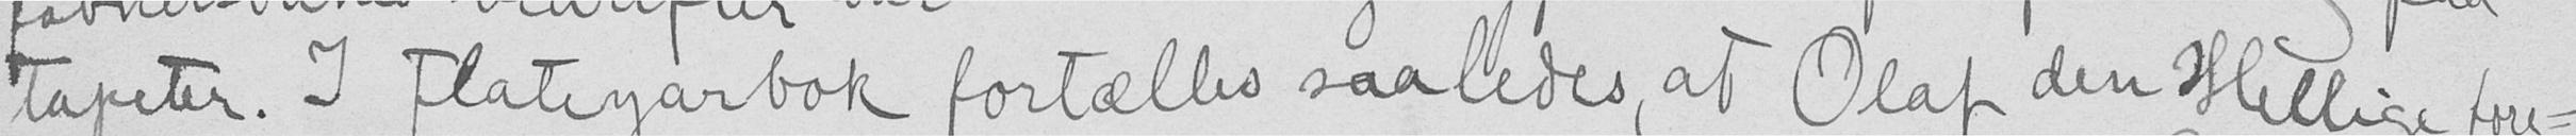trapeter. I flategarbok fortælles saaledes, at Olaf den Hellige fore-
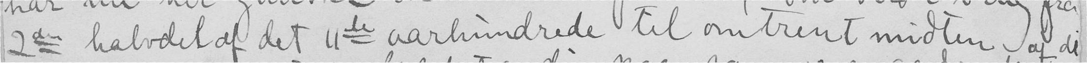2den halvdel af det 11de aarhundrede til omtrent midten af det
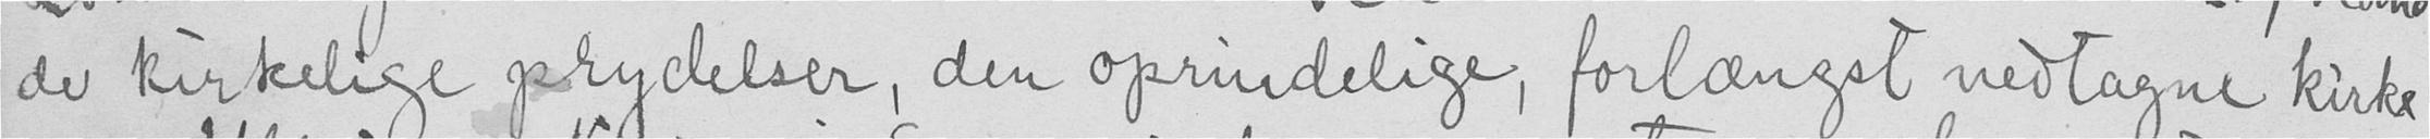de kirkelige prydelser, den oprindelige, forlængst nedtagne kirke
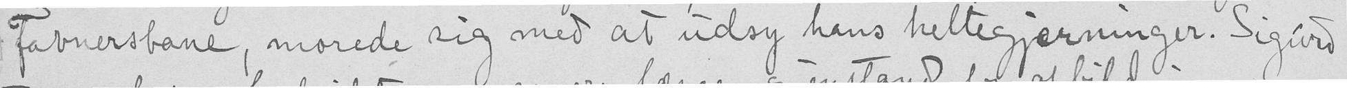Favnersbane, morede sig med at udsy hans heltegjerninger. Sigurd
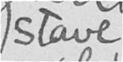stave
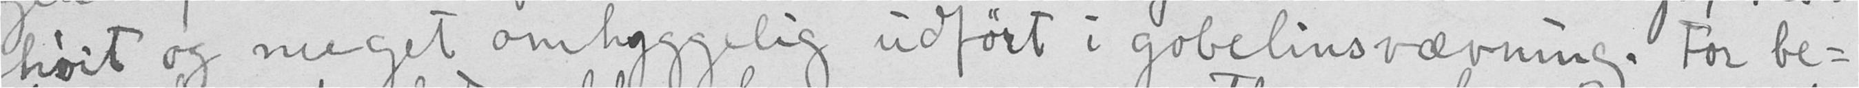høit og meget omhyggelig udført i gobelinsvævning. For be-
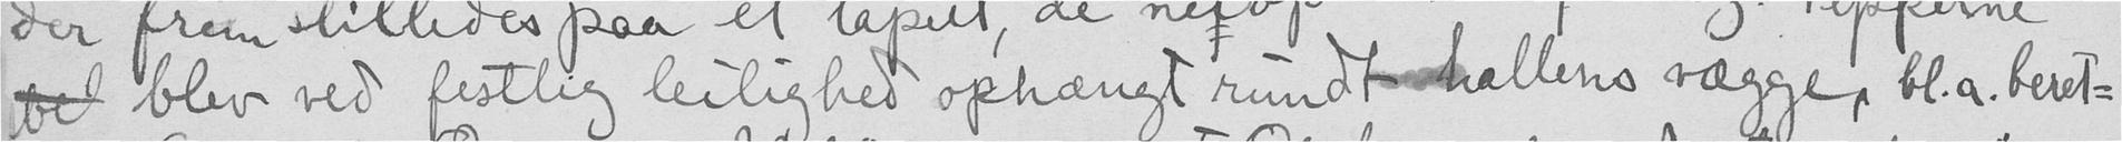blev ved festlig leilighed ophængt rundt hallens vægge, bl.a beret-
